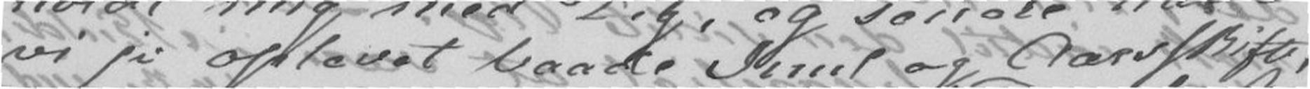vi jo oplevet baade Juul og Aarsskifte,
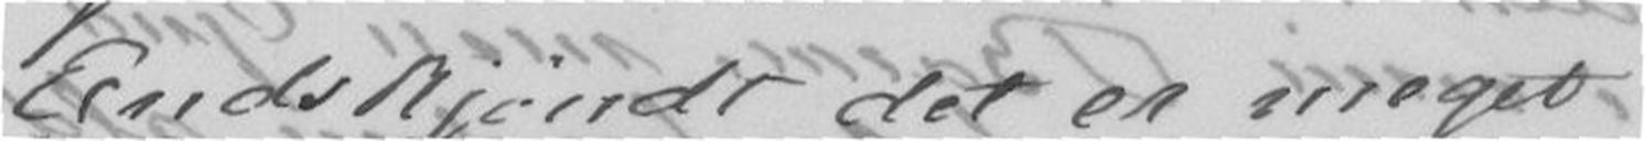Endskjøndt det er meget
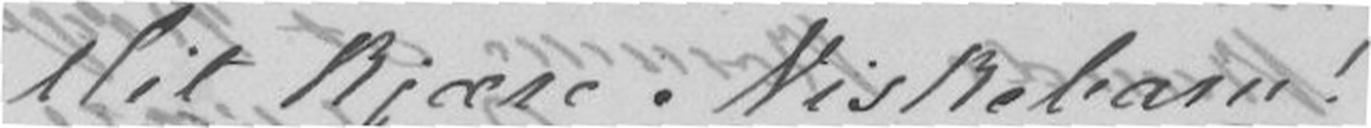Mit kjære Niskebarn!
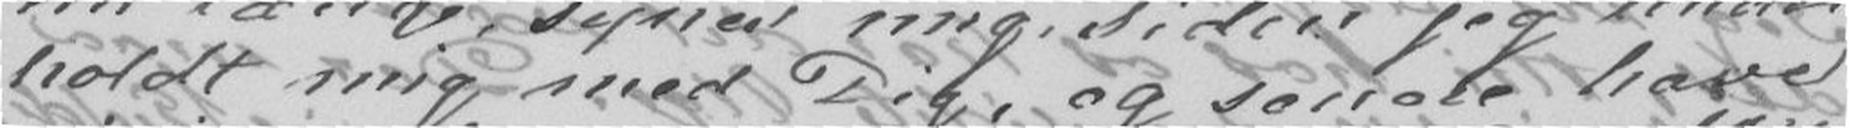holdt mig med Dig, og senere have
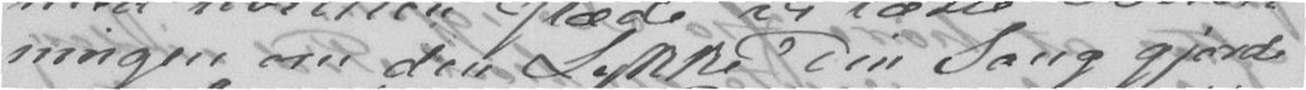ningen om den Lykke Din Sang gjorde
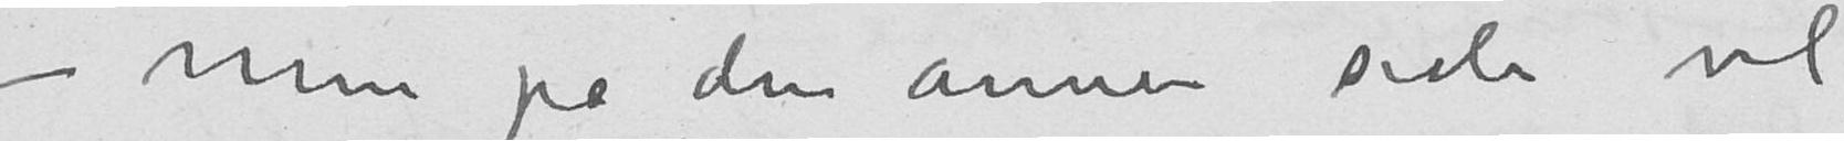– Men på den annen side vil
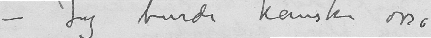– Jeg burde kanske osså
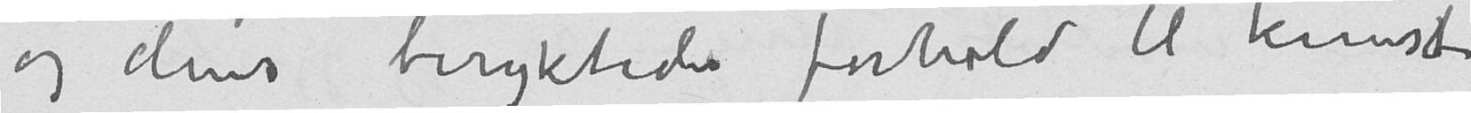og dens beryktede forhold til Kunst
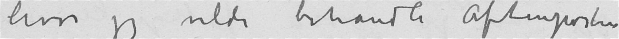hvor jeg vilde behandle Aftenposten
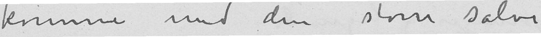komme med en større salve
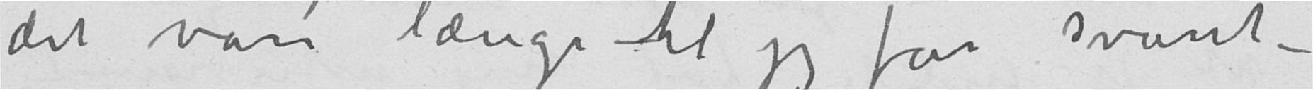det vare længe –til jeg får svaret –
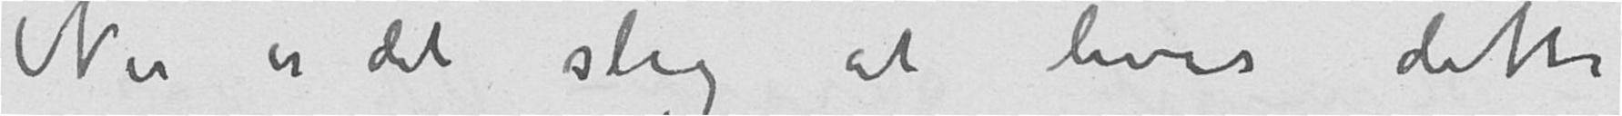Nu er det slig at hvis dette
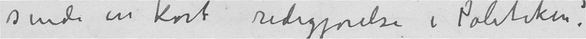sende en kort redegjørelse i Politiken?
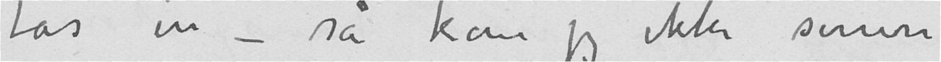tas in – så kan jeg ikke senere
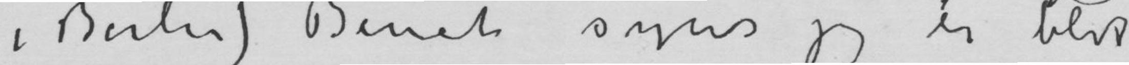i Berlin) Benet syns jeg er blit
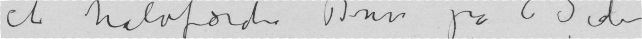et halvfærdi Brev på 6 Sider
